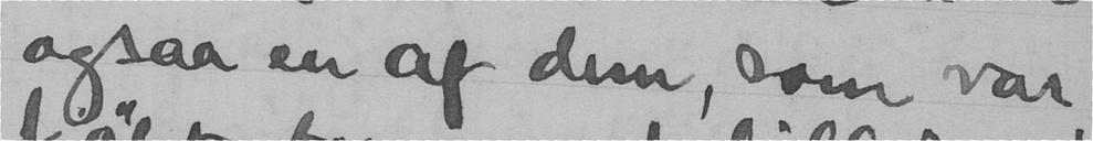ogsaa en af dem, som var
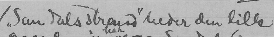("Sandalsstrand" heder den lille
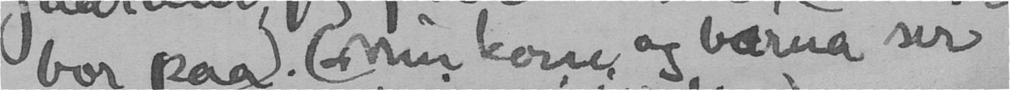bor paa). (Min kore og barna ser
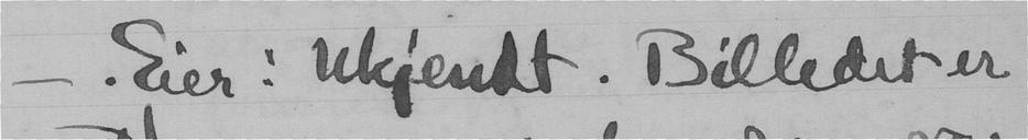- Eier: ukjendt. Billedet er
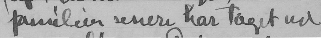familien senere har taget ud
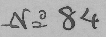No 84
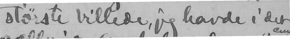største billede, jeg havde i det
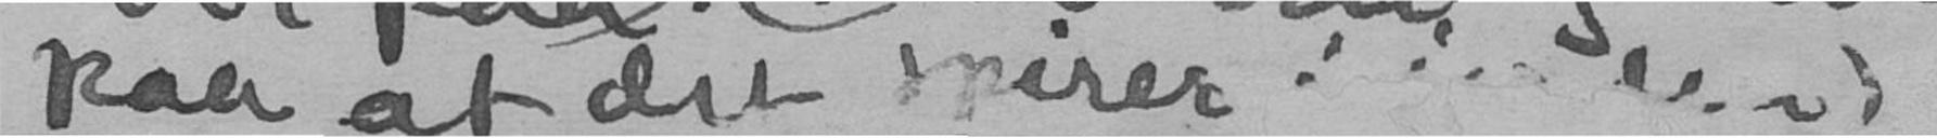paa at det spirer!
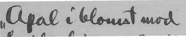"Apal i blomst mod
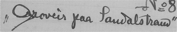"Groveir paa Sandalstrand"
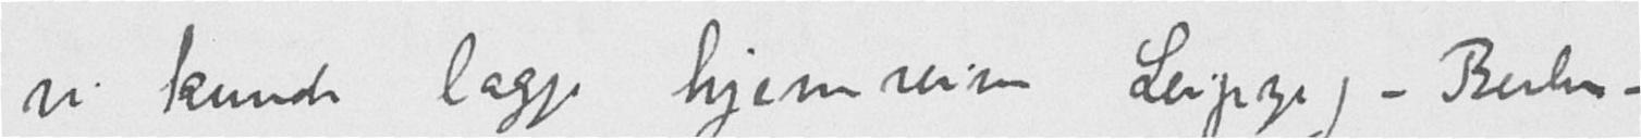vi kunde lægge hjemreisen Leipzig-Berlin-
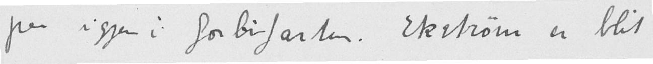paa igjen i forbifarten. Ekstrøm er blit
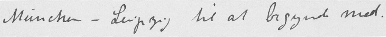München-Leipzig til at begynde med.
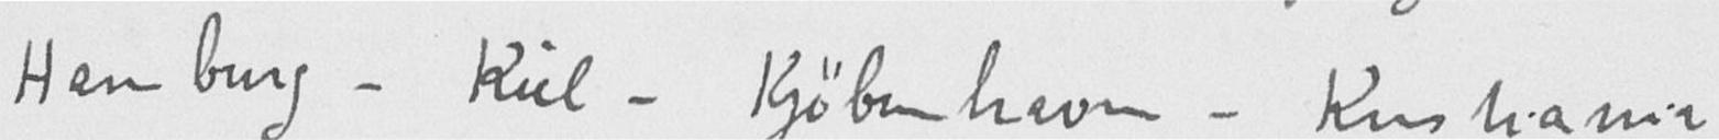Hamburg-Kiel-Kjøbenhavn-Kristiania
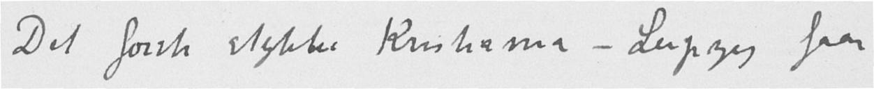Det første stykke Kristiania-Leipzig faar
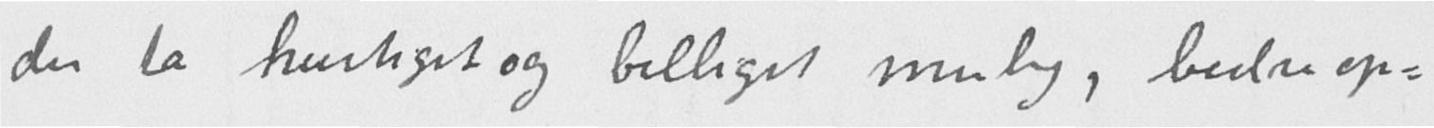du ta hurtigst og billigst mulig, bedre op-
